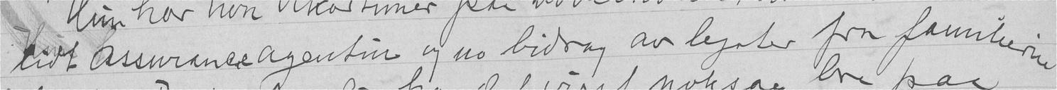lidt Assuranceagentur og no bidrag av legater fra familierne
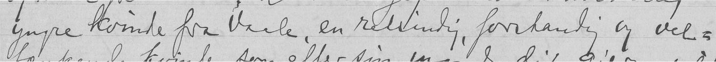yngre kvinde fra Vaale, en retsindig, forstandig og vel-
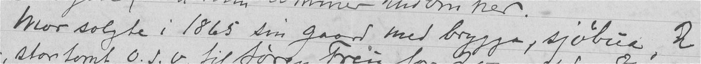Mor solgte i 1865 sin gaard med brygge, sjøbu, 2
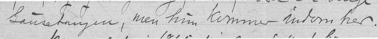Gausetangen, men hun kommer indom her.
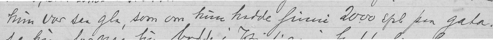hun var saa gla, som om hun hadde finni 2000 spd paa gata.
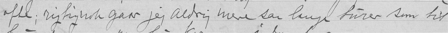ofte; rigtignok gaar jeg aldrig mere saa lange turer som til
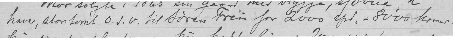haver, stor tomt o.s.v. til Søren Treu for 2000 spd. - 8000 kroner.
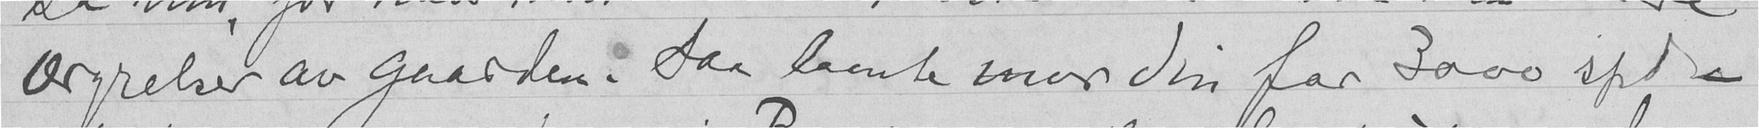Ærgrelser av gaarden. Saa laante mor din far 3000 spd -
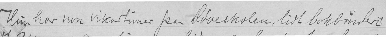Hun har noen vikartimer paa Døveskolen, lidt bokbinderi
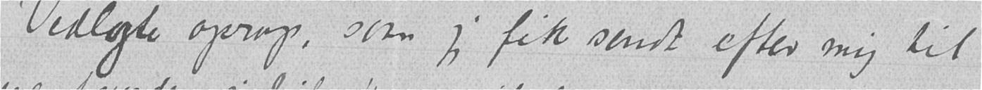Vedlagte oprop, som jeg fik sendt efter mig til
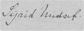Sigrid Undset.
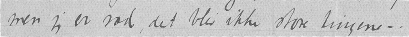men jeg er ræd, det blir ikke store tingene –.
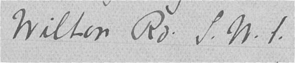Wilton Rd. S.H.T.
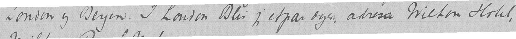London og Bergen. I London Blir jeg etpar dager, adresse Wilton Hotel,
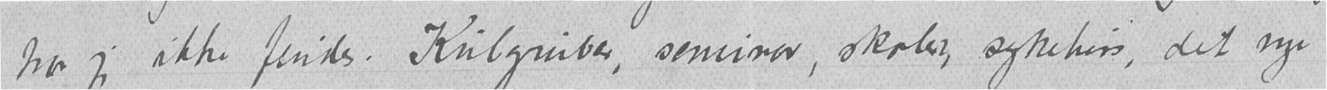tror jeg ikke findes. Kulgruber, seminar, skoler, sykehus, det nye
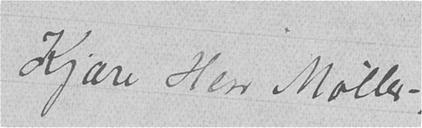Kjære Herr Møller-,
